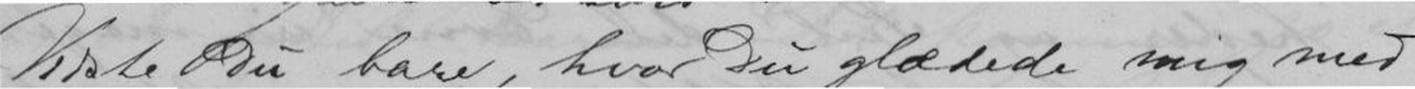Vidste Du bare, hvor Du glædede mig med
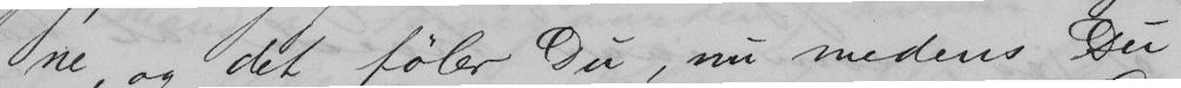ne, og det føler Du, nu medens Du
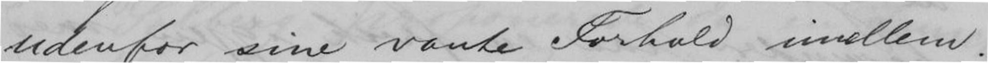udenfor sine vante Forhold imellem.
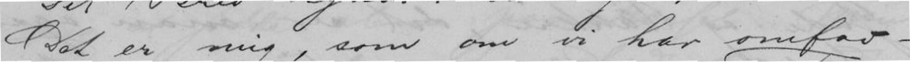Det er mig, som om vi har omfav-
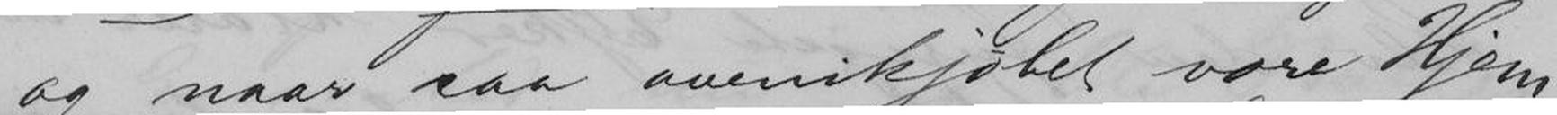og naar saa ovenikjøbet vore Hjem
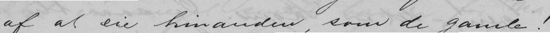af at eie hinanden, som de gamle!
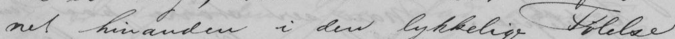net hindanden i den lykkelige Følelse
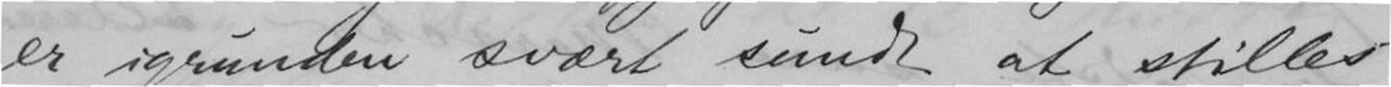er igrunden svært sundt at stilles
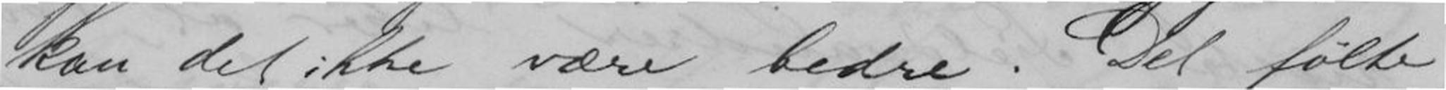kan det ikke være bedre. Det følte
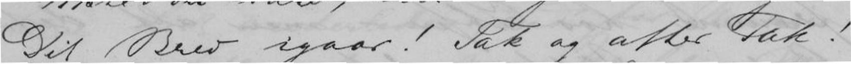Dit Brev igaar! Tak og atter Tak!
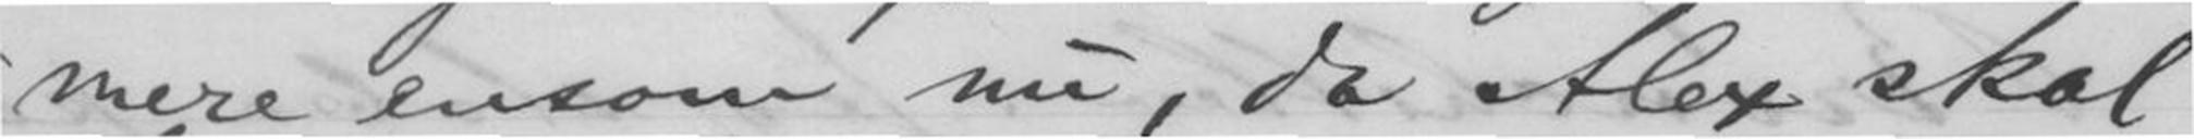mere ensom nu, da Alex skal
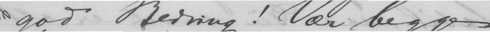god Bedring! Vær begge
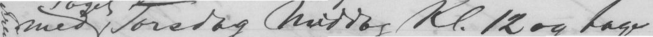med Torsdag Middag kl. 12 og tage
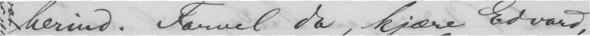herind. Farvel da kjære Edvard,
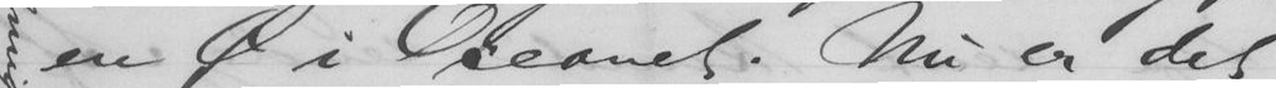en Ø i Oceanet. Nu er det
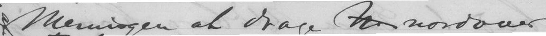Meningen at drage N nordover
